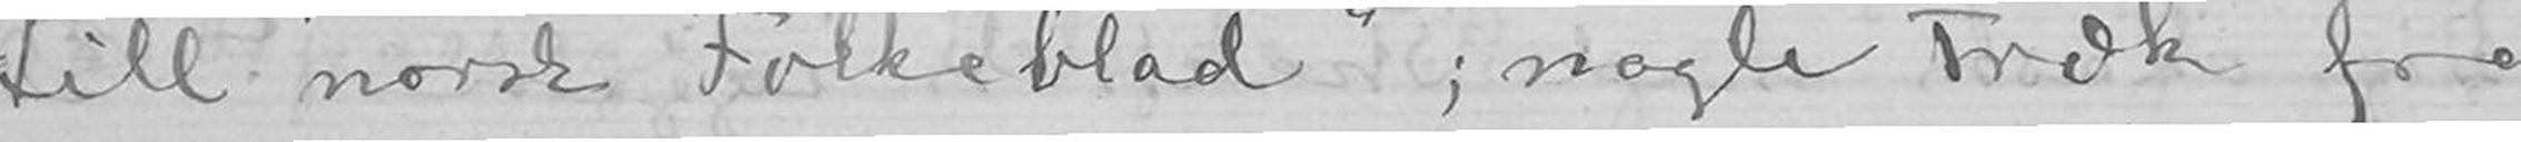till «norsk Folkeblad»; nogle Træk fra
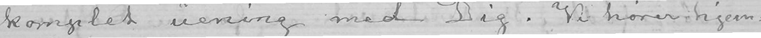komplet uening med Dig. Vi hører hjem-
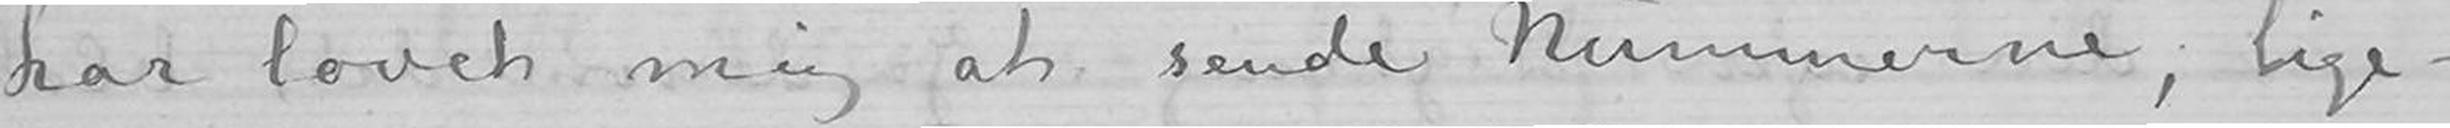har lovet mig at sende Nummerne; lige-
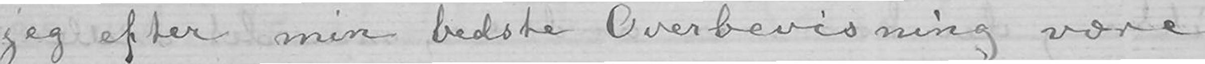jeg efter min bedste Overbevisning være
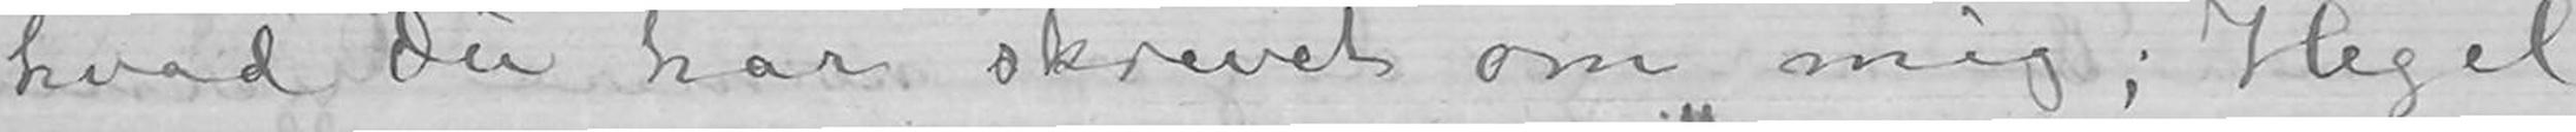hvad Du har skrevet om mig; Hegel
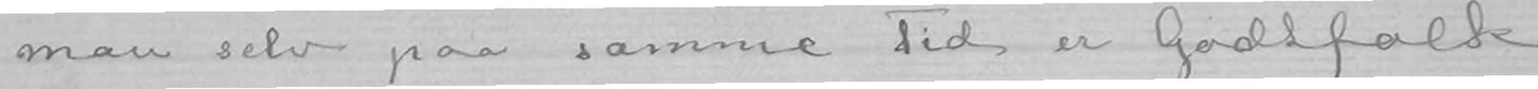man selv paa samme Tid er Godtfolk
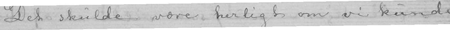Det skulde være herligt om vi kunde
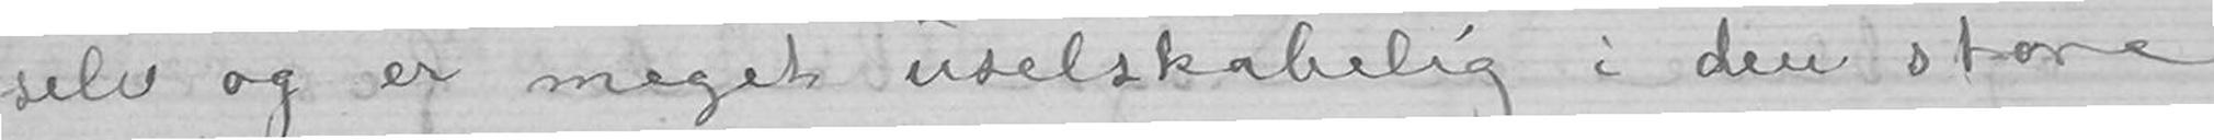selv og er meget uselskabelig i den store
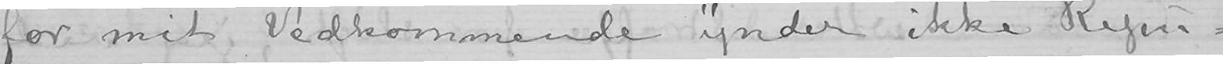for mit Vedkommende ynder ikke Repu-
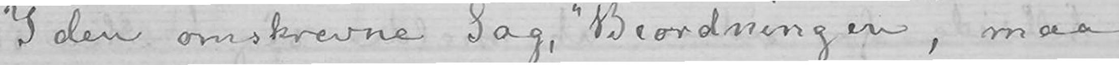I den omskrevne Sag, «Beordningen», maa
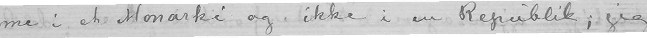me i et Monarki og ikke i en Republik; jeg
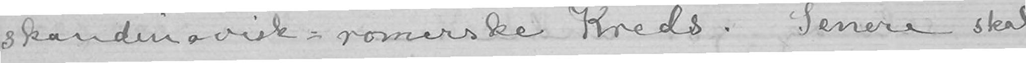skandinavisk-romerske Kreds. Senere skal
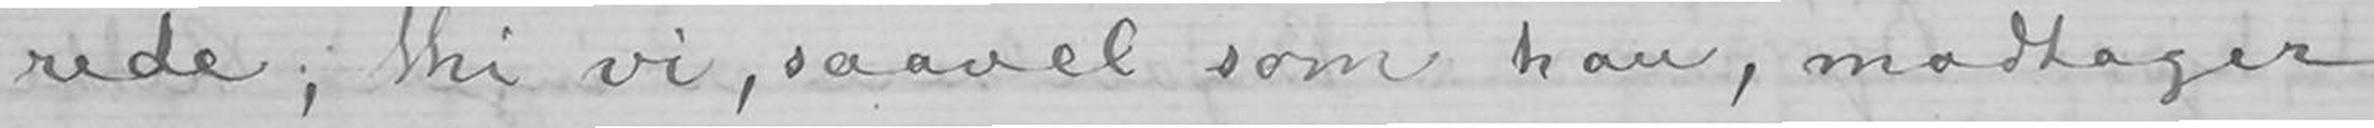rede; thi vi, saavel som han, modtager
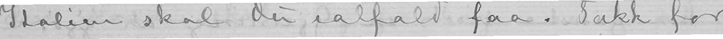Italien skal Du ialfald faa. Takk for
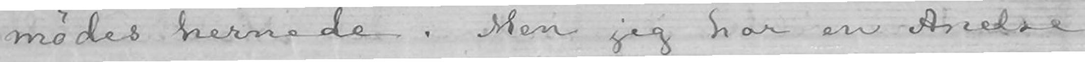mødes hernede. Men jeg har en Anelse
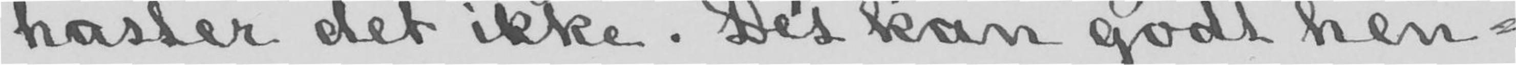haster det ikke. Det kan godt hen-
